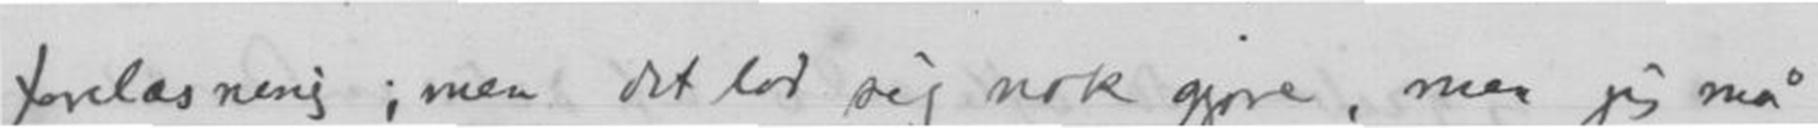forelæsning; men det lod sig nok gjøre, men jeg må
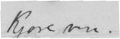Kjære ven.
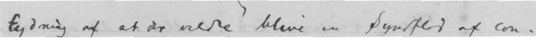tydning af at der vilde blive en syndflod af con-
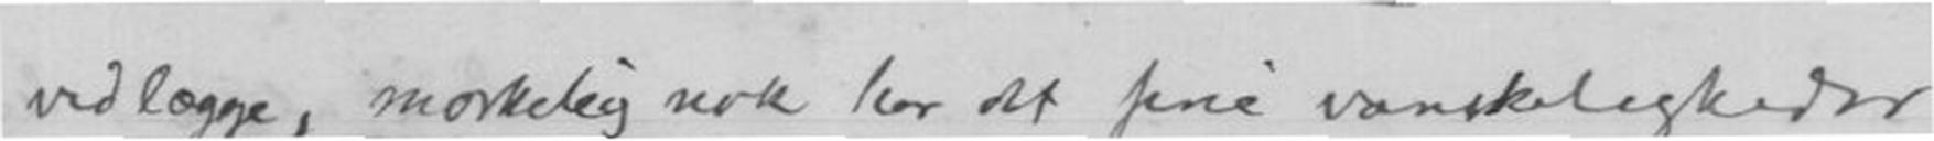vedlegge, mærkelig nok har det sine vanskeligheder
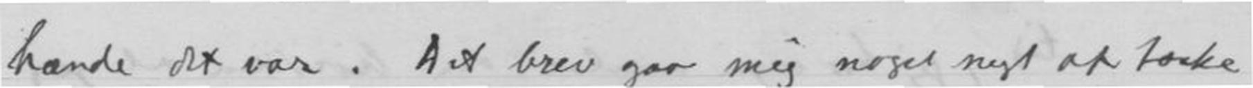hænde det var. Det brev gav mig noget nyt at tænke
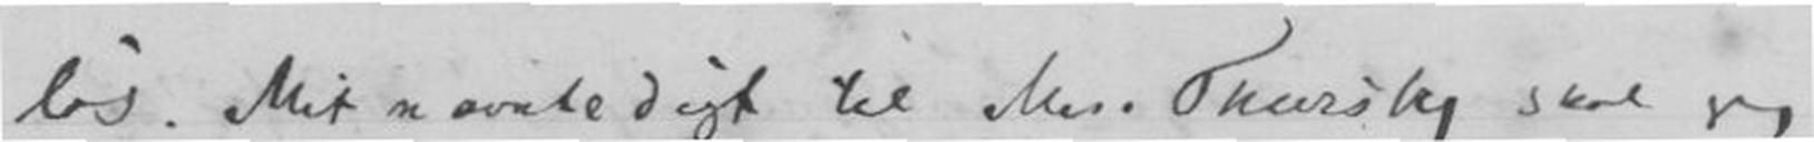løs. Mit nævnte digt til Mrs. Thursly skal jeg
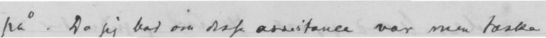på. Da jeg bad om disse assitaner var min tanke
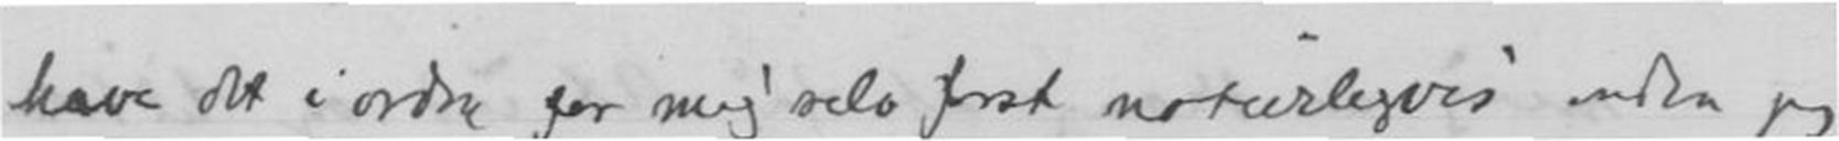have det i orden for mig selv først naturligvis inden jeg
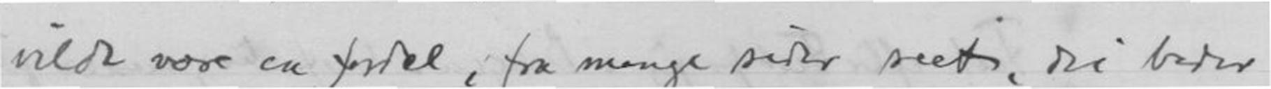vilde være en fordel, fra mange sider seet, du beder
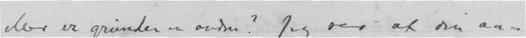der er grunder en orden? Jeg ser af din an-
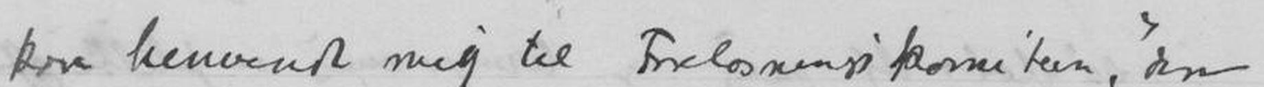kan henvende mig til Forelesningskomiteen, "den
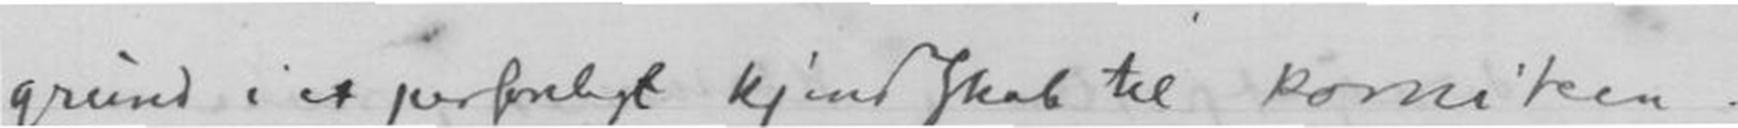grund i et personligt kjendskab til komiteen
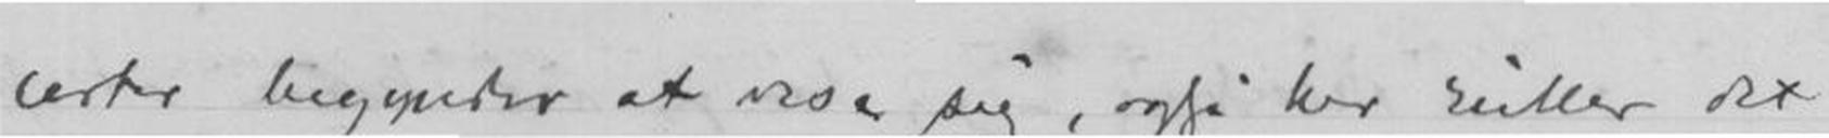certer begynder at vise sig, også her ruller det
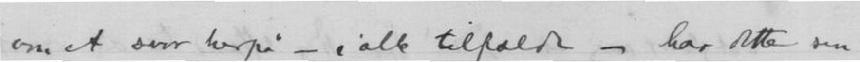om et svar herpå - i alle tilfælde - har dette sin
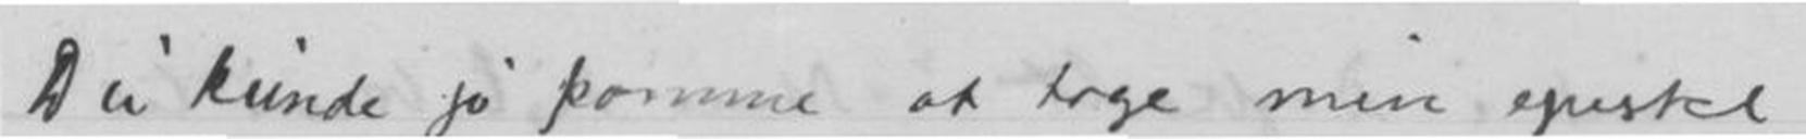Du kunde jo komme at tage min epistel
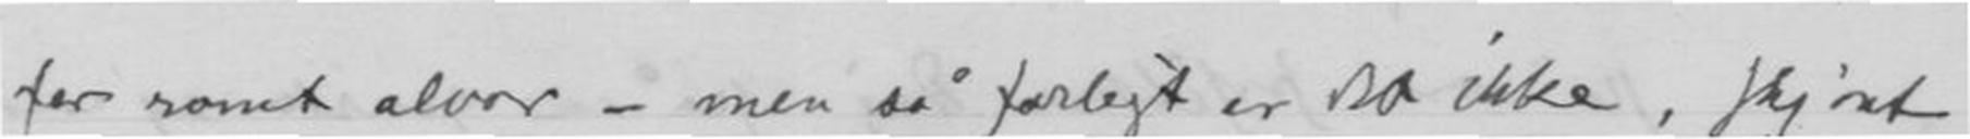for ramt alvor - men så farligt er det ikke, skjønt
